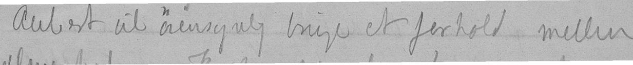Aubert vil øiensynlig bringe et forhold mellem
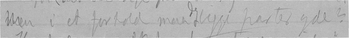Men i et forhold maa dog begge parter yde?
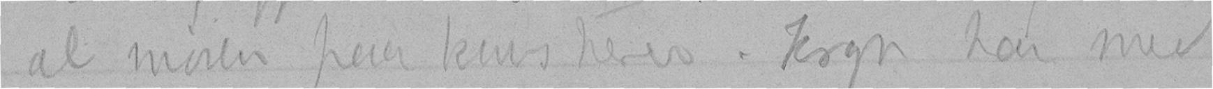al møien paa kunstneren. Krogh har med
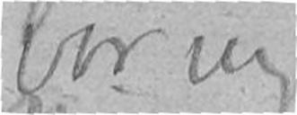vor nye
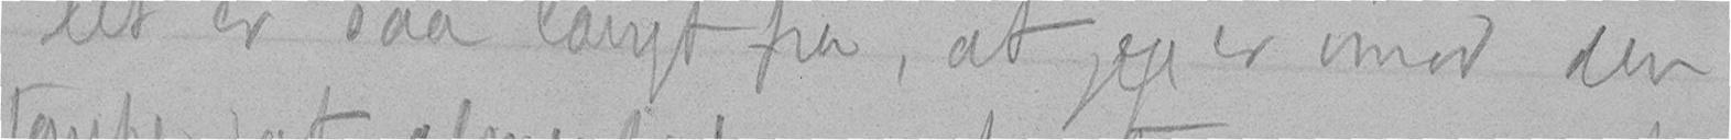det er saa langt fra, at jeg er imod den
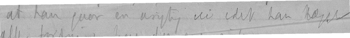at han gaar en urigtig vei idet han lægger
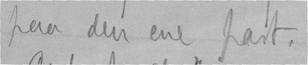paa den ene part.
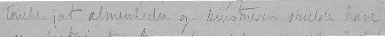tanke at almenheden og kunstneren skulde have
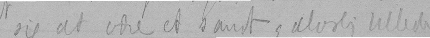sig at være et sandt og alvorlig billede
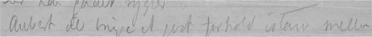Aubert vil bringe et godt forhold istand mellem
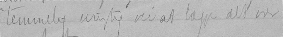temmelig urigtig vei at lægge det over
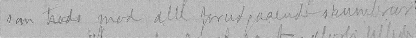som trods mod alle forudgaaende skumlerier
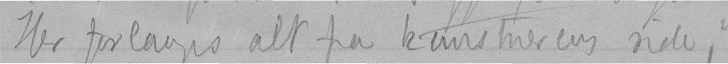Her forlanges alt fra kunstnerens side,
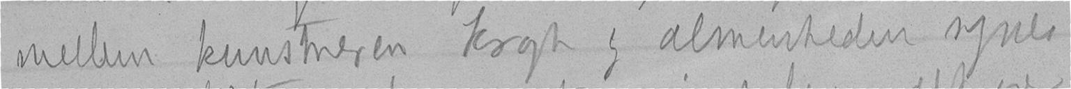mellem kunstneren Krogh og almenheden synes
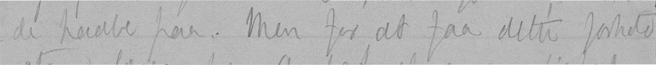de haabe paa. Men for at faa dette forhold
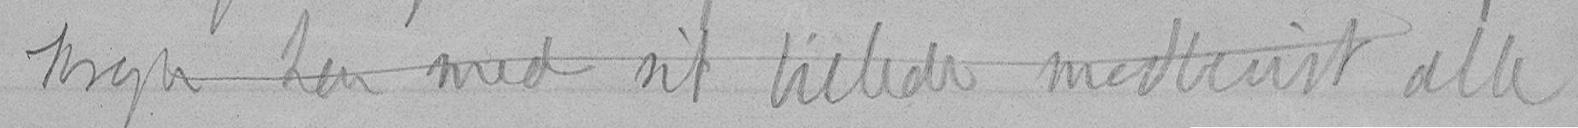Krogh har med sit billede modbevist alle
